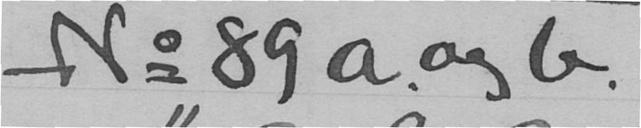No 89 a og b
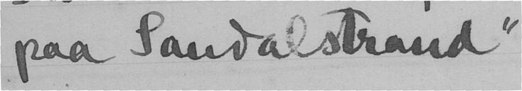paa Sandalstrand"
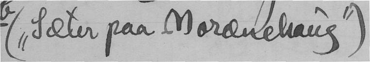("Sæter paa Morænehaug")
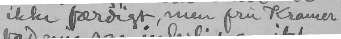ikke færdigt, men fru Kramer
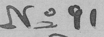No 91
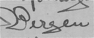Bergen
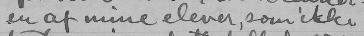en af mine elever, som ikke
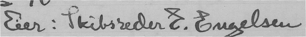Eier: Skibsreder E. Engelsen
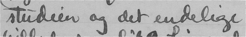studien og det endelige
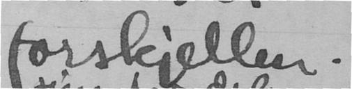forskjellen.
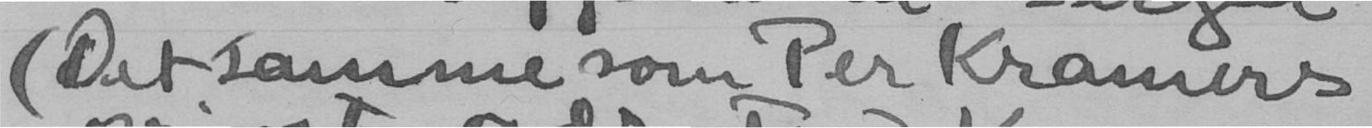(Det samme som Per Kramers
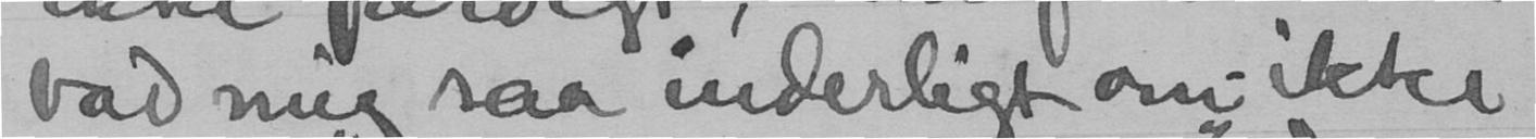bad mig saa inderligt om ikke
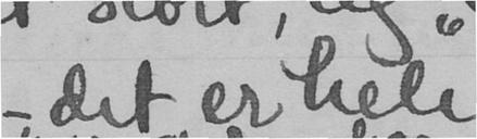- de er hele
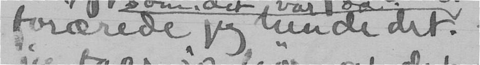forærede jeg hende det
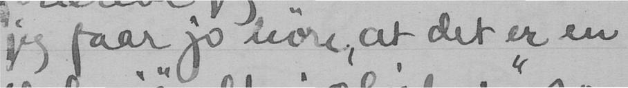jeg faar jo høre, at det er en
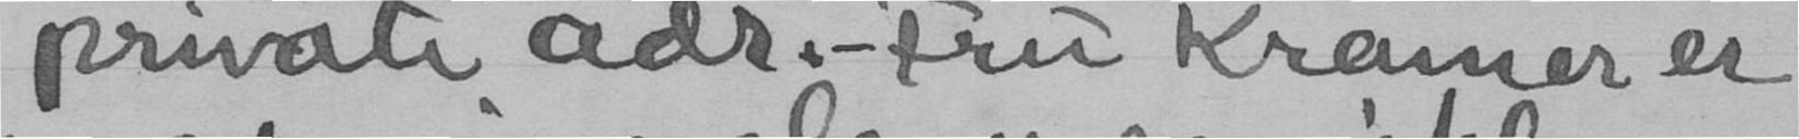private adr. - Fru Kramer er
